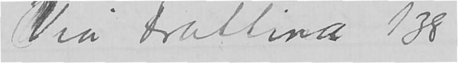Via Frattina 138
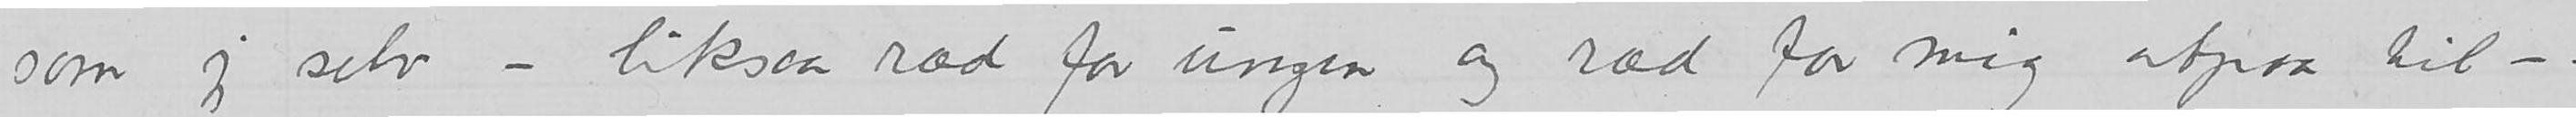som jeg selv – liksom ræd for ungen og ræd for mig atpaa til –.
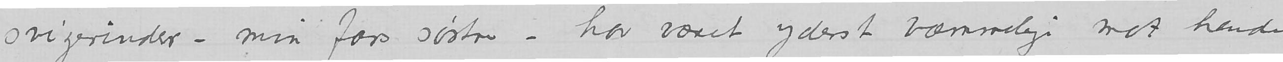svigerinder – min fars søstre – har været yderst væmmelige mot hende
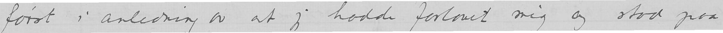først i anledning av at jeg hadde forlovet mig og stod paa
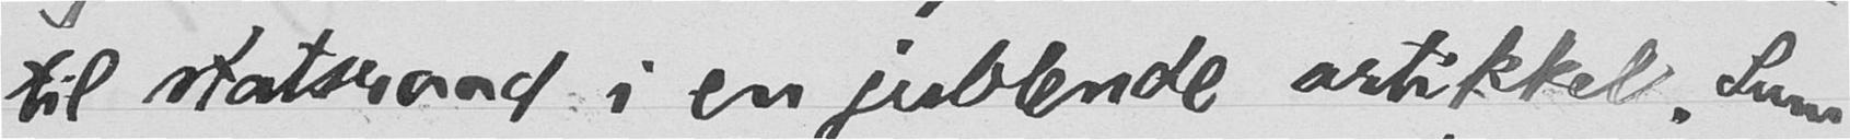til statsraad i en jublende artikkel. Sum-
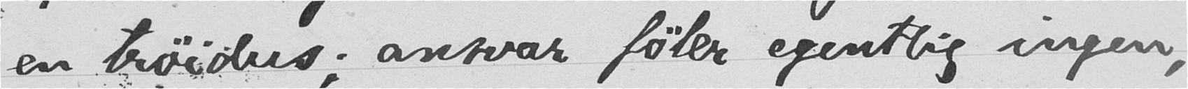en trøidus; ansvar føler egentlig ingen,
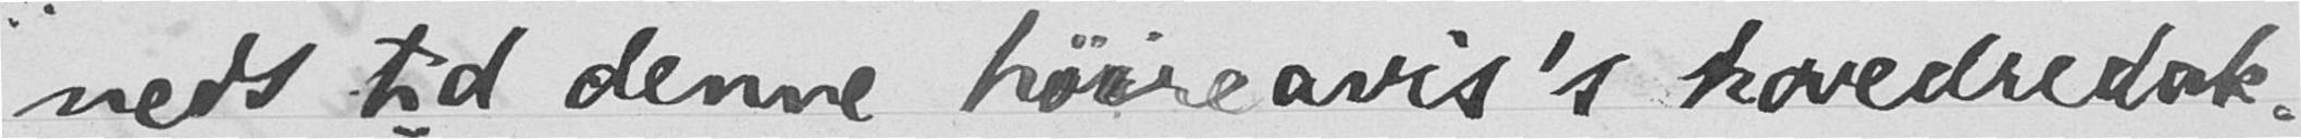neds tid denne høireavis’s hovedredak-
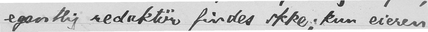egentlig redaktør findes ikke; kun eieren
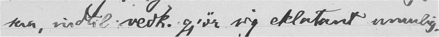saa, indtil vedk. gjør sig eklatant umulig.
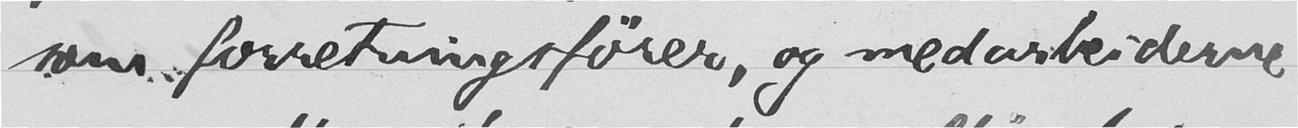som forretningsfører, og medarbeiderne
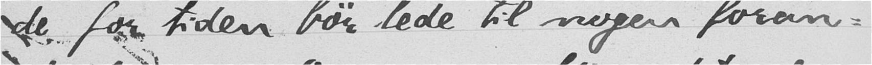de for tiden bør lede til nogen foran-
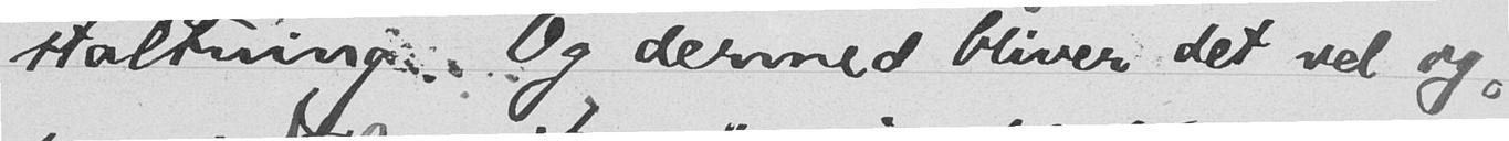staltning. Og dermed bliver det vel og-
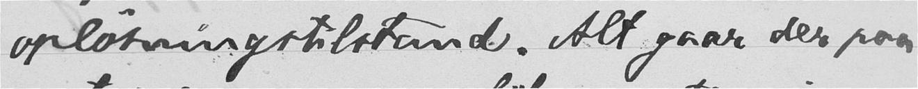opløsningstilstand. Alt gaar der paa
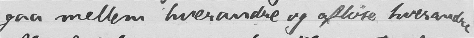gaa mellem hverandre og afløse hverandre
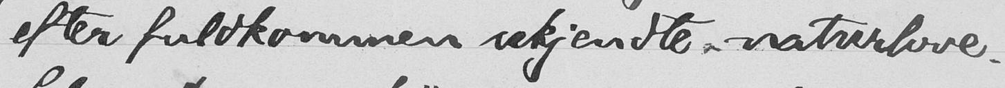efter fuldkommen ukjendte naturlove.
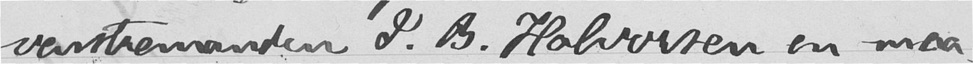venstremanden S. B. Halvorsen en maa-
